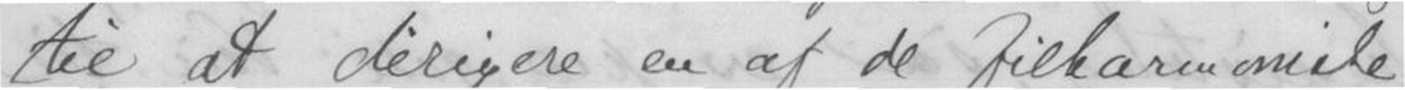til at dirigere en af de filharmoniske
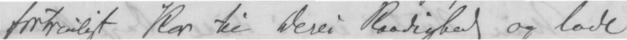fortræfligt Kor til Deres Raadighed og lade
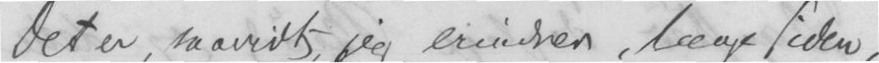Det er, saavidt jeg erindrer, længe siden,
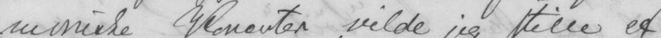moniske Koncerter vilde jeg stille et
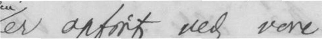er opført ved vore
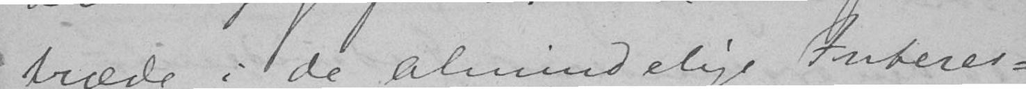træde i de almindelige Interes-
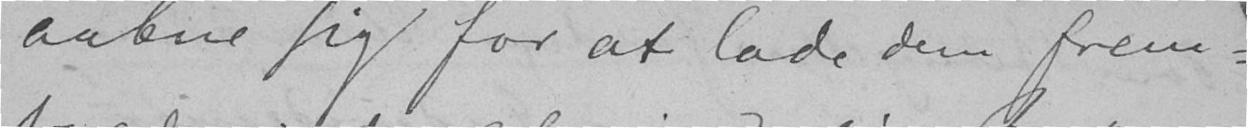aabne sig for at lade dem frem-
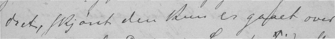dret, skjønt den kun er gaaet over
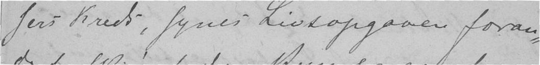sers Kreds, synes Livsopgaven foran-
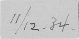11/12. 34.
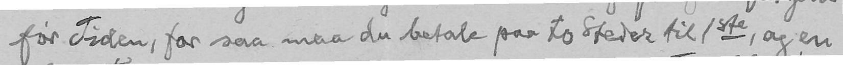før Tiden, for saa maa du betale paa to Steder til 1ste, og en
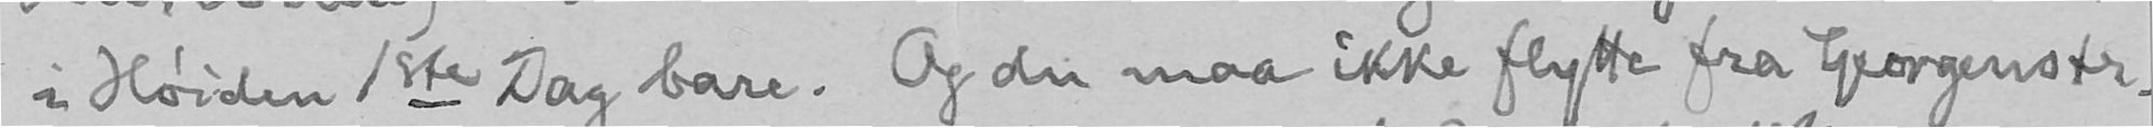i Høiden 1ste Dag bare. Og du maa ikke flytte fra Georgenstr.
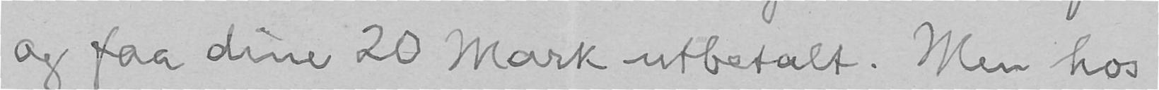og faa dine 20 Mark utbetalt. Men hos
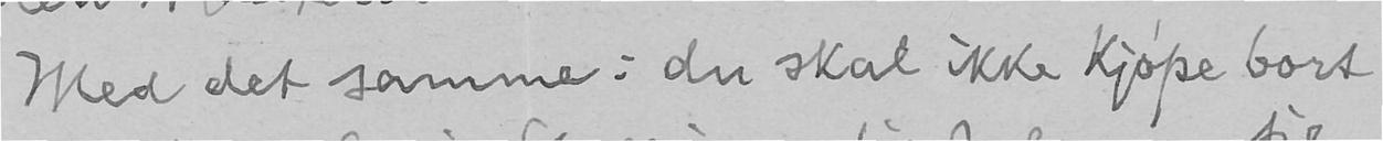Med det samme: du skal ikke kjøpe bort
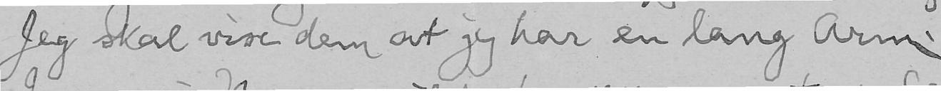Jeg skal vise dem at jeg har en lang Arm.
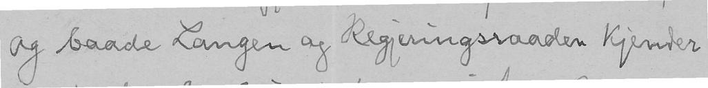og baade Langen og Regjeringsraaden kjender
